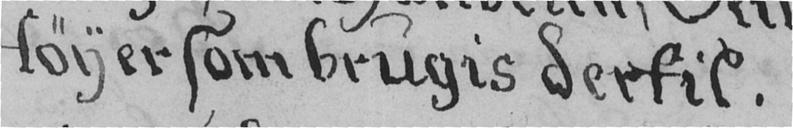tøyer som brugis dertil.
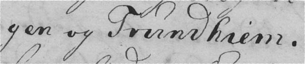gen og Trundhiem.
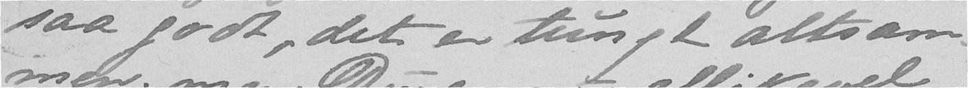saa godt, det er tungt altsam-
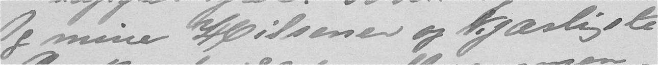Og mine Hilsener og kjærligste
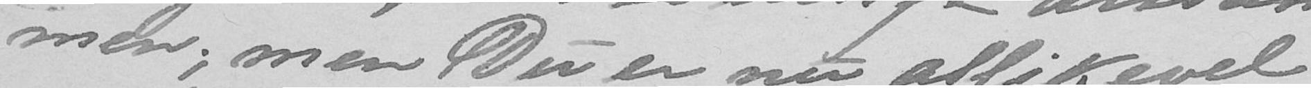men; men Du er nu allikevel
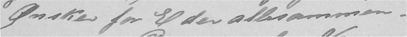Ønsker for Eder allesammen.
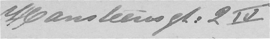Hansteensgt. 2 IV
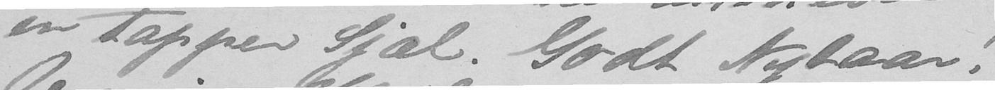en tapper Sjæl. Godt Nytaar!
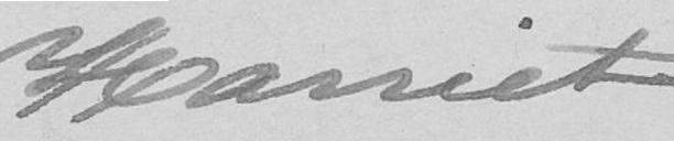Harriet
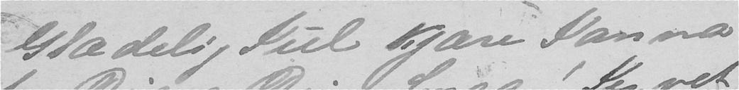Glædelig Jul kjære Janna
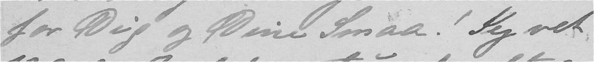for Dig og Dine Smaa! Jeg vet
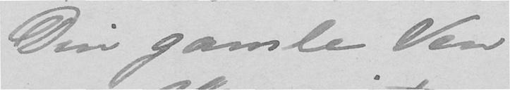Din gamle Ven
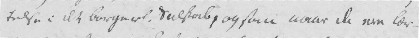telse i det borgerl. Selskab, og som naar de ere lær-
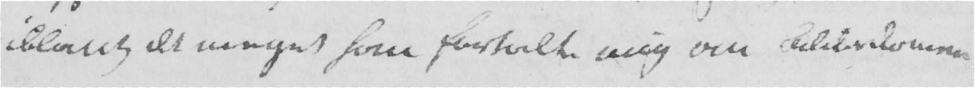iblant det meget han fortalte mig om Alderdommen
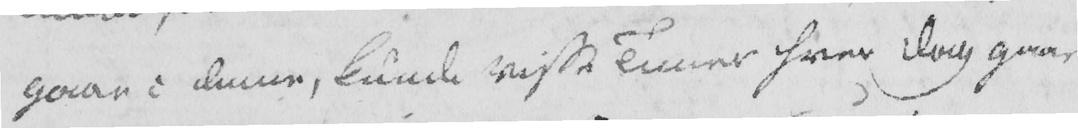gaae i denne, kunde visse Timer hver Dag gaae
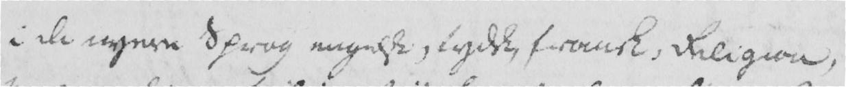i de nyere Sprog engelsk, tydsk, fransk, Religion,
